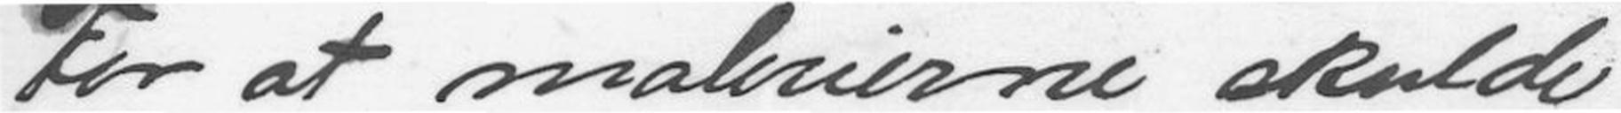For at malerierne skulde
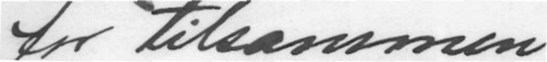for tilsammen
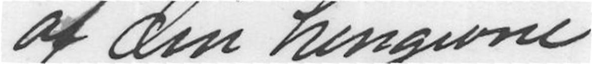af din hengivne
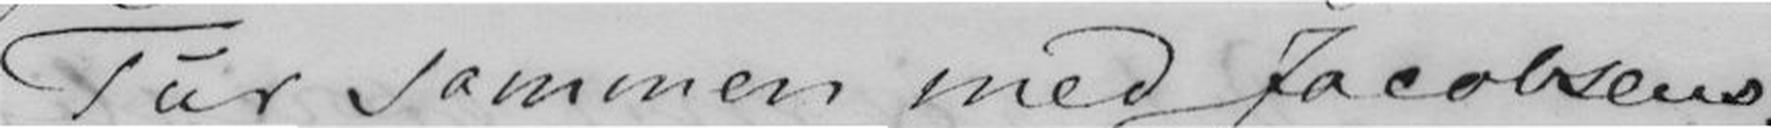Tur sammen med Jacobsens.
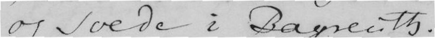og svede i Bayreuths.
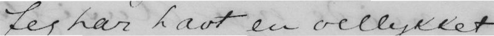Jeg har havt en vellykket
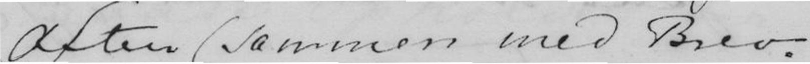Aften (sammen med Brev-
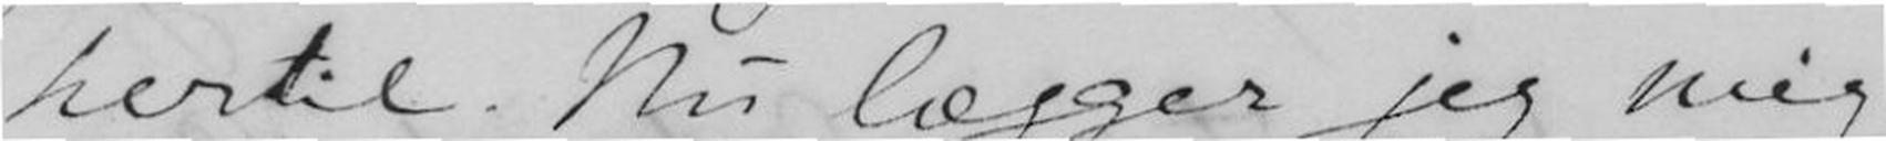hertil. Nu legger jeg mig
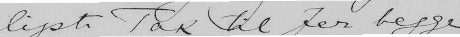ligste Tak til Jer begge
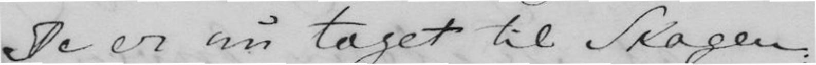De er nu taget til Skagen;
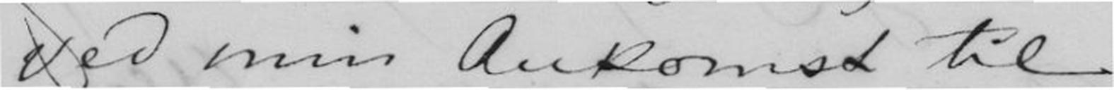ved min Ankomst til
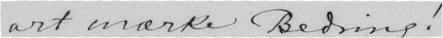art mærke Bedring!
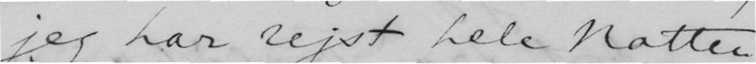jeg har rejst hele Natten
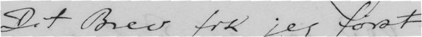Dit Brev fik jeg først
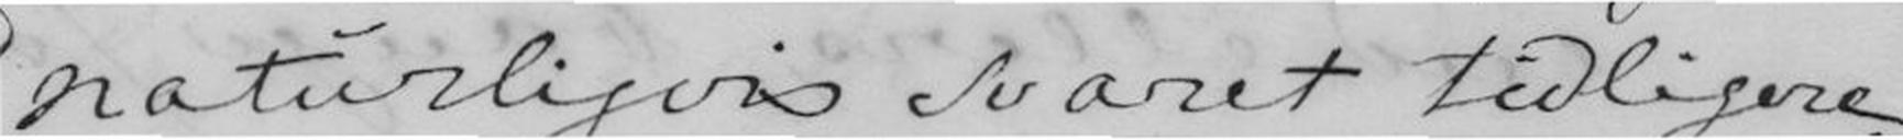naturligvis svaret tidligere.
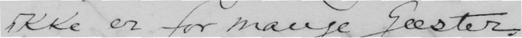ikke er for mange Gæster.
